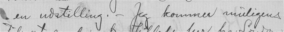en udstilling. - Jge kommer muligens
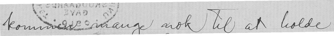kommen mange nok til at holde
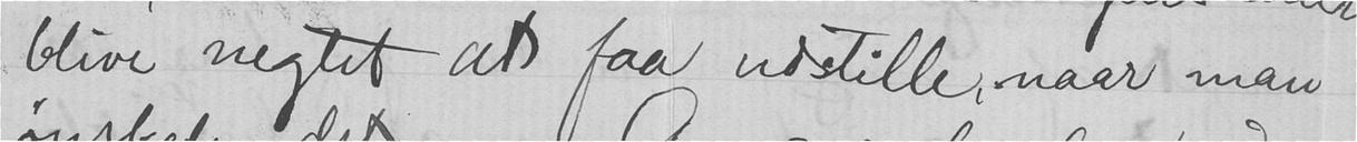blive negtet at faa udstille, naar man
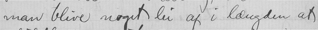man blive noget lei af i længden at
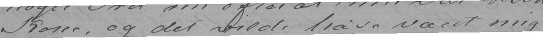Kone, og det vilde have været mig
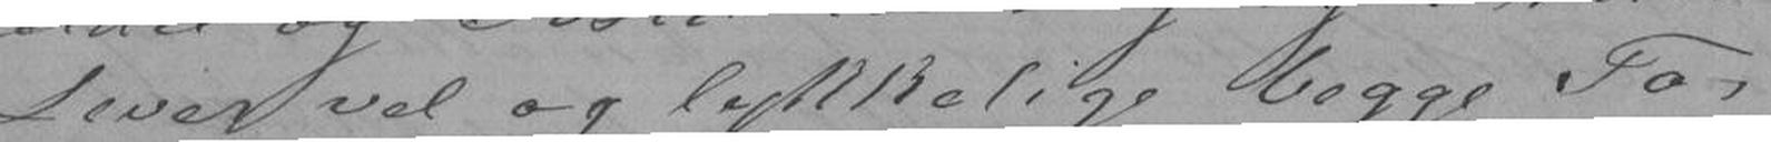Lever vel og lykkelige begge To,
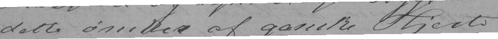dette ønskes af ganske Hjerte
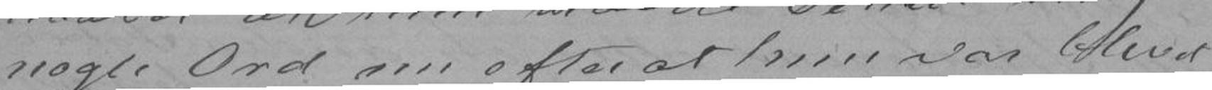nogle Ord nu efterat hun var blevet
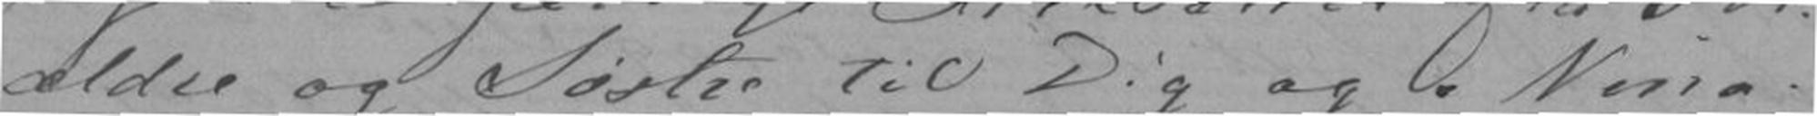ældre og Søstre til Dig og Nina
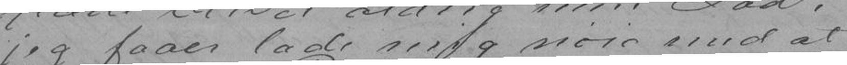jeg faaer lade mig nøie med at
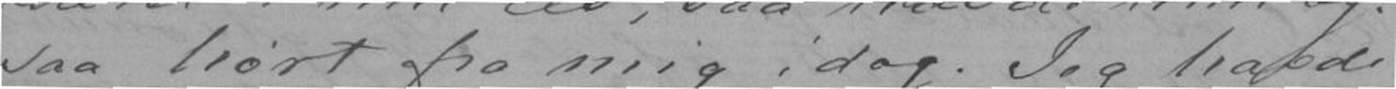saa hørt fra mig idag. Jeg havde
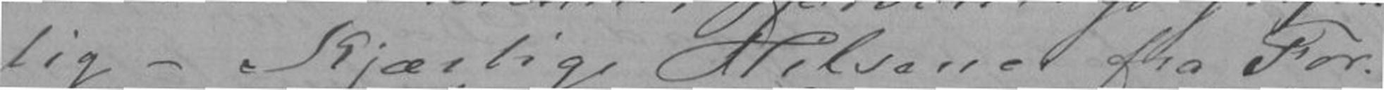lig - Kjærlig Hilsener fra For-
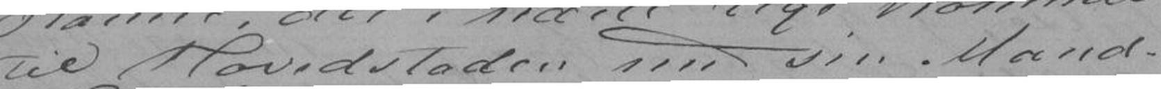til Hovedstaden med sin Mand.
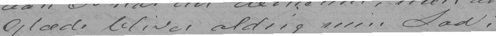Glæde bliver aldrig min Lod;
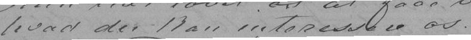hvad der kan interessere os.
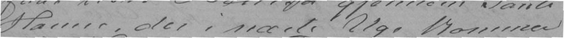Hanne, der i næste Uge kommer
